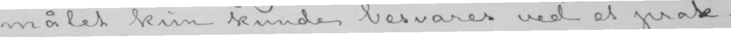målet kun kunde besvares ved et prak-
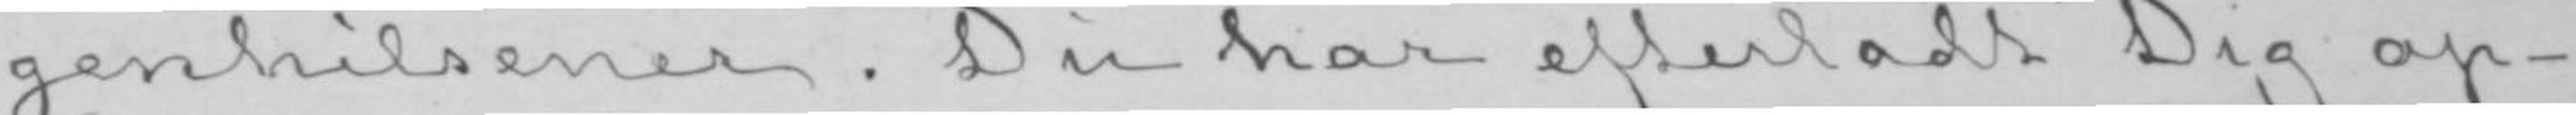genhilsener. Du har efterladt Dig op-
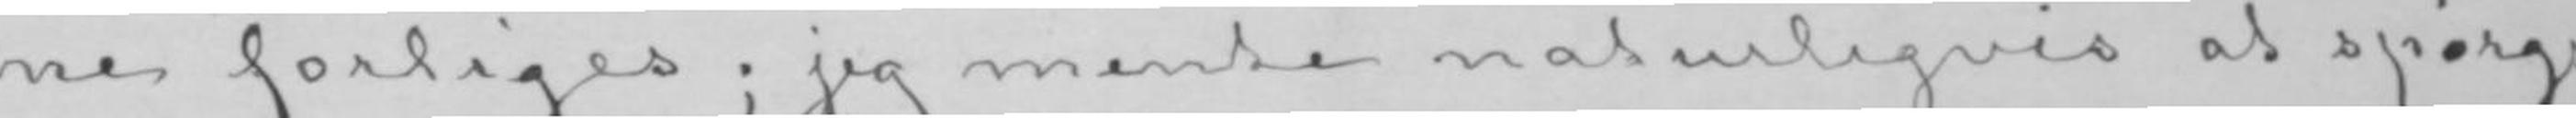ne forliges; jeg mente naturligvis at spørgs-
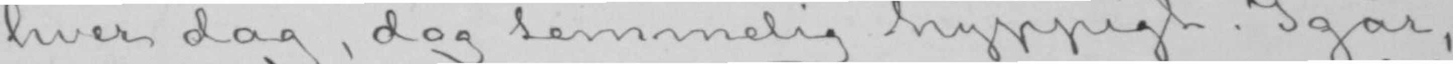hver dag, dog temmelig hyppigt. Igår,
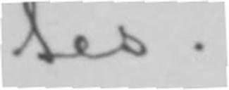tes.
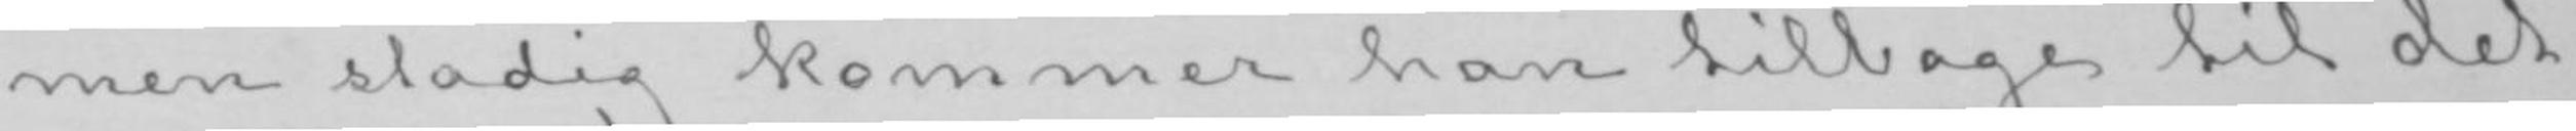men stadig kommer han tilbage til det
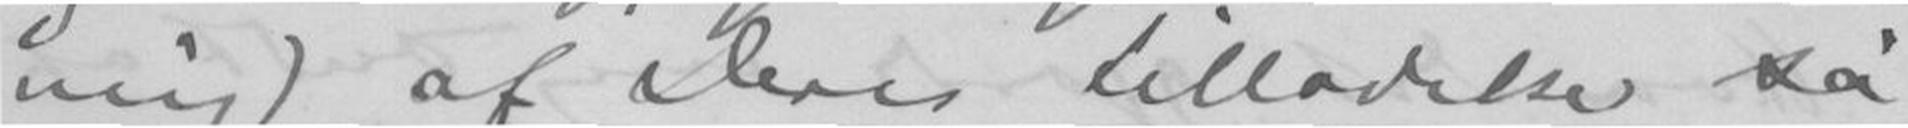mig af Deres tilladelse så
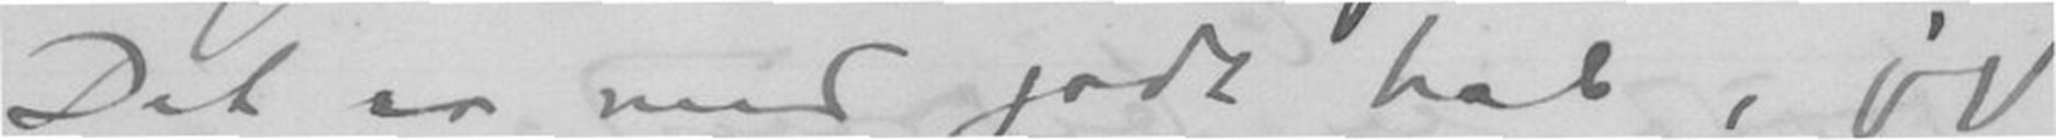Det er med godt håb, jeg
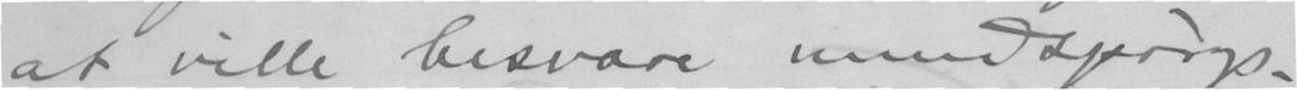at ville besvare mine spørg-
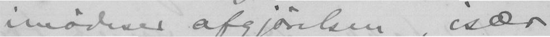imødeser afgjørelsen, især
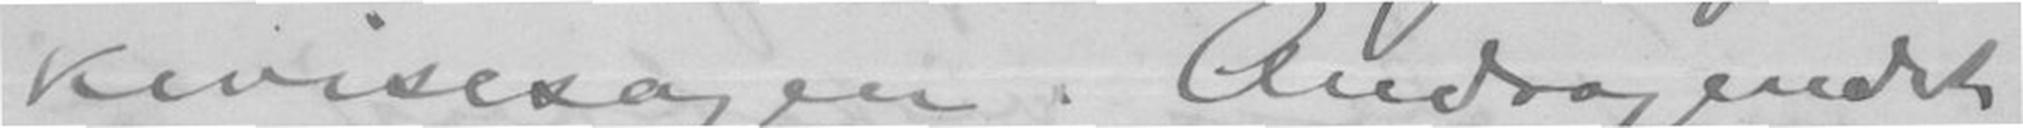kevisesagen. Andragendet
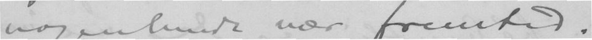nogenlunde nær fremtid.
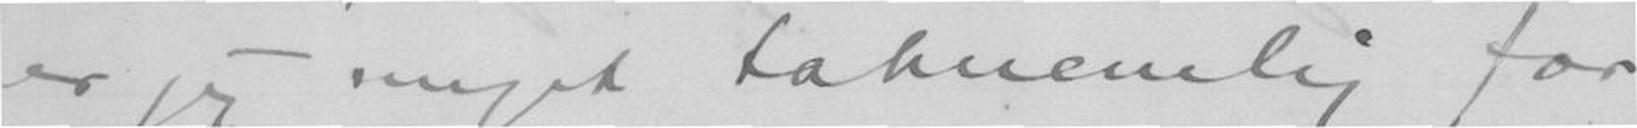er jeg meget taknemlig for
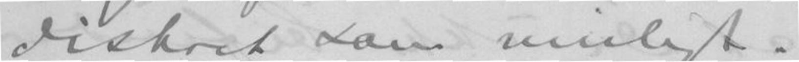diskret som muligt.
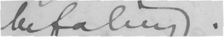befaling.
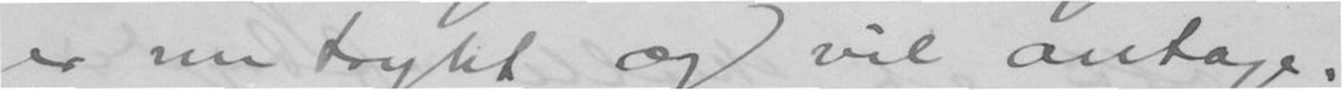er nu trykt og vil antage-
